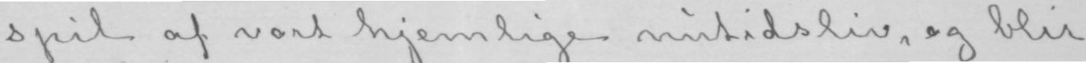spil af vort hjemlige nutidsliv, og blir
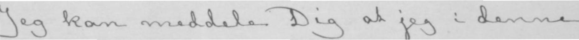Jeg kan meddele Dig at jeg i denne
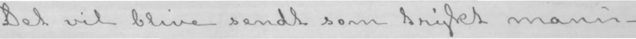Det vil blive sendt som trykt manu-
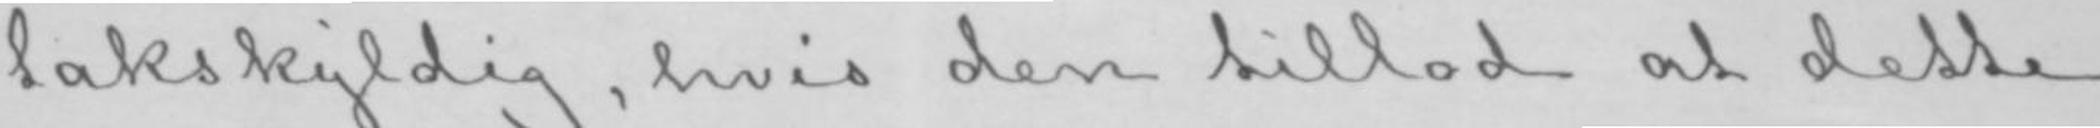takskyldig, hvis den tillod at dette
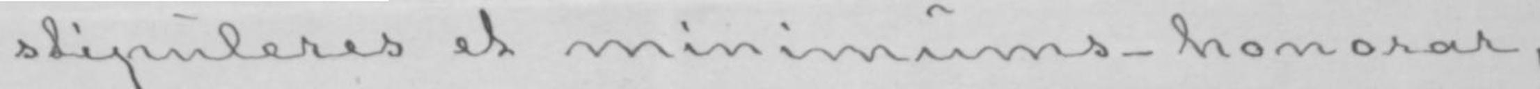stipuleres et minimums-honorar,
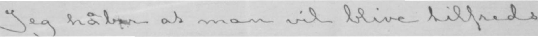Jeg håber at man vil blive tilfreds
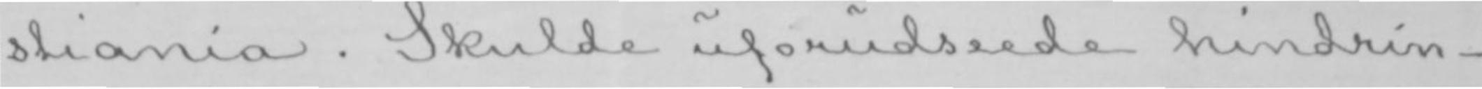stiania. Skulde uforudseede hindrin-
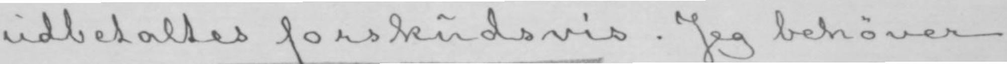udbetaltes forskudsvis. Jeg behøver
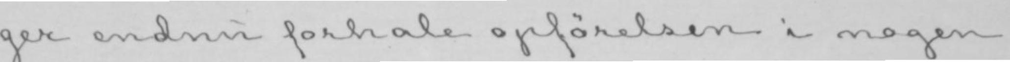ger endnu forhale opførelsen i nogen
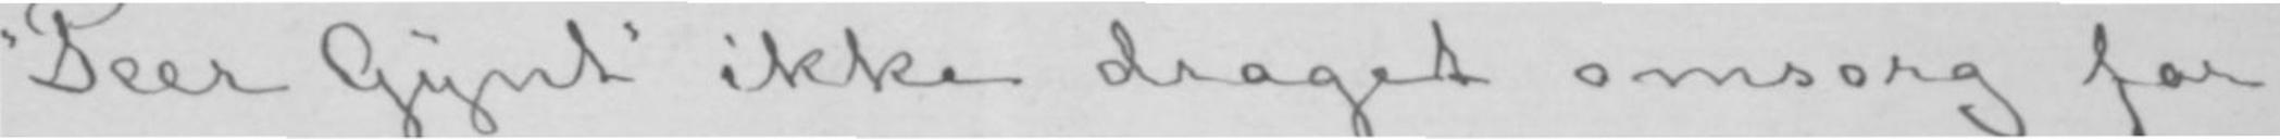«Peer Gynt» ikke draget omsorg for
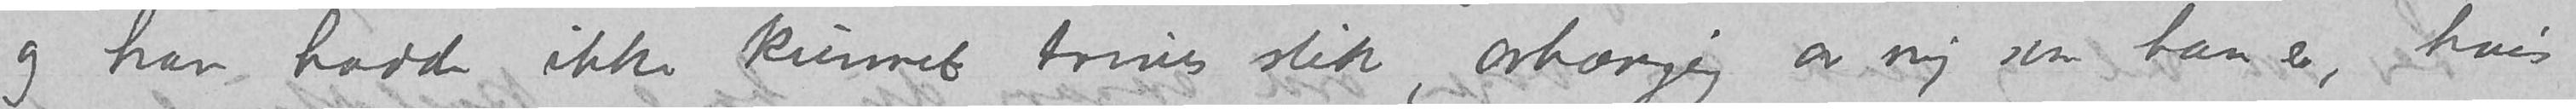og han hadde ikke kunnet trives slik, avhængig av mig som han er, hvis
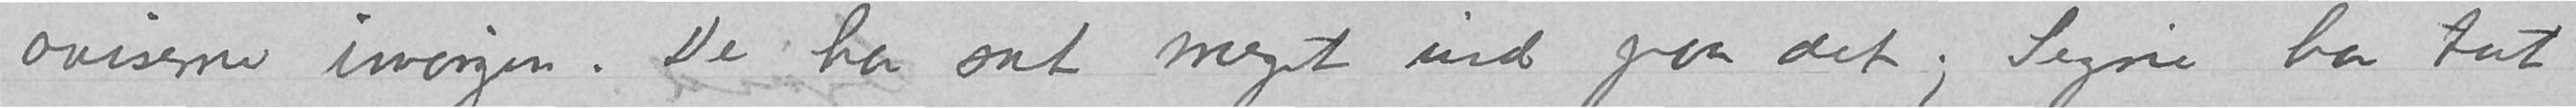aviserne imorgen. De har sat meget ind paa det; Signe har tat
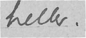heller.
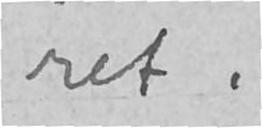ret.
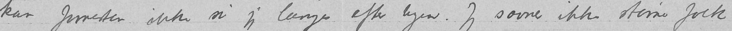kan forresten ikke si jeg længes efter byen. Jeg savner ikke større folk
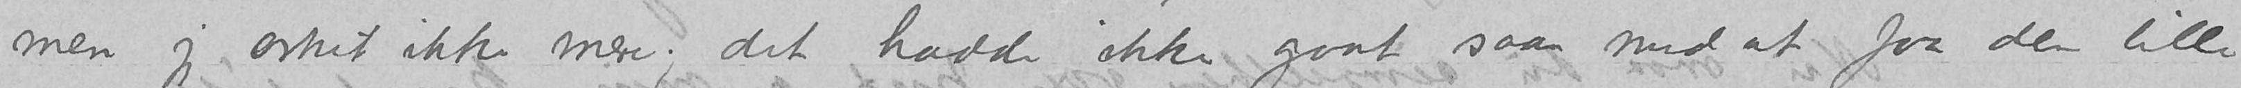men jeg orket ikke mere; det hadde ikke gaat saan med at faa den lille
